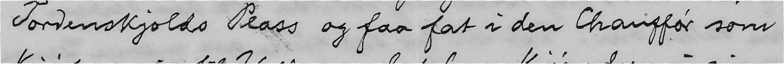Tordenskjolds Plass og faa fat i den Chauffør som
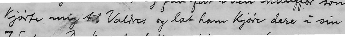kjørte mig til Valdres og lat ham kjøre dere i sin
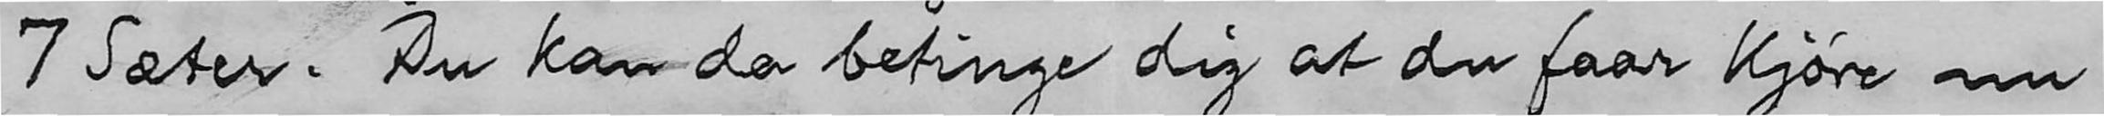7 Sæter. Du kan da betinge dig at du faar kjøre nu
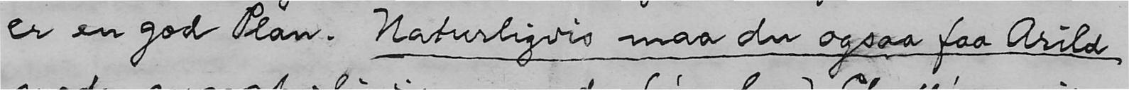er en god Plan. Naturligvis maa du ogsaa faa Arild
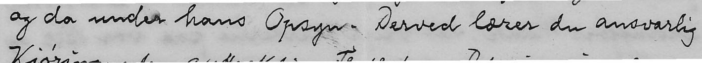og da under hans Opsyn. Derved lærer du ansvarlig
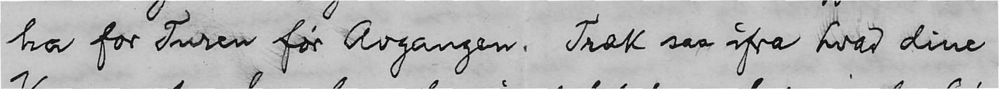ha for Turen før Avgangen. Træk saa ifra hvad dine
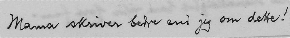Mama skriver bedre end jeg om dette!
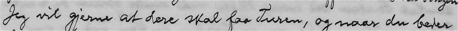Jeg vil gjerne at dere skal faa Turen, og naar du beder
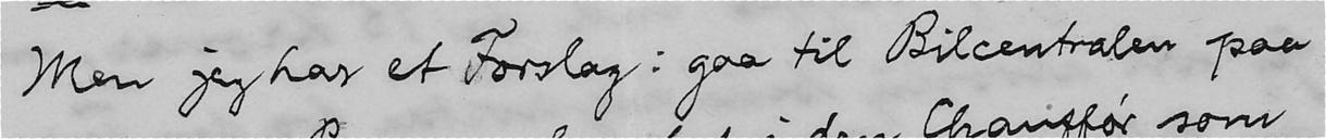Men jeg har et Forslag: gaa til Bilcentralen paa
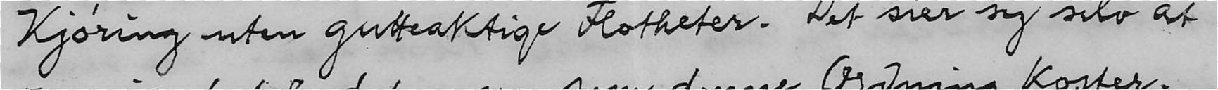Kjøring uten gutteaktige Flotheter. Det sier sig selv at
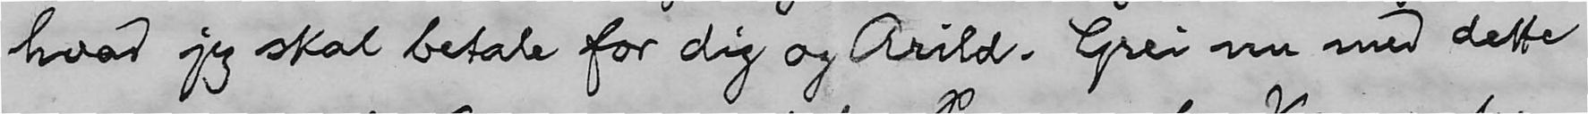hvad jeg skal betale for dig og Arild. Grei nu med dette
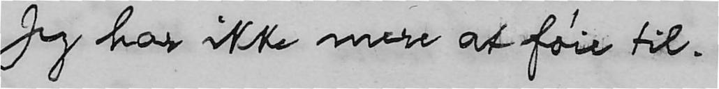Jeg har ikke mere at føie til.
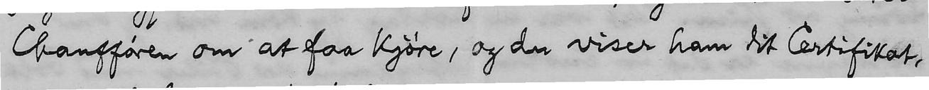Chaufføren om at faa kjøre, og du viser ham dit Certifikat,
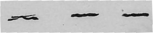- - -
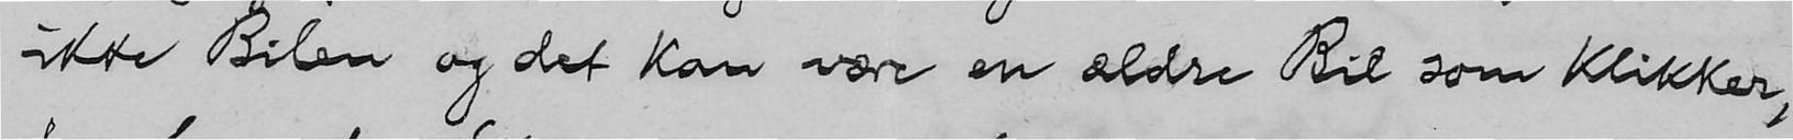ikke Bilen og det kan være en ældre Bil som klikker,
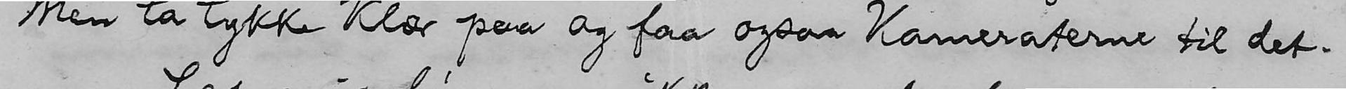Men ta tykke Klær paa og faa ogsaa Kameraterne til det.
